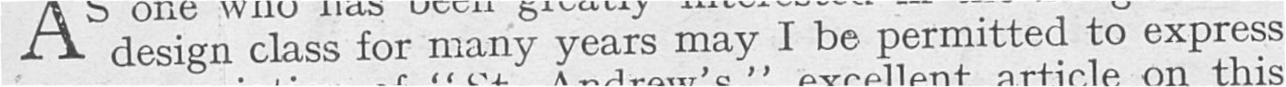design class for many years may I be permitted to express
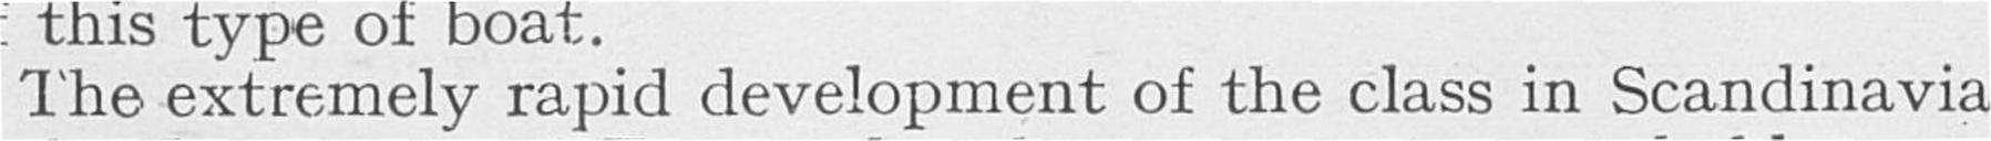The extremely rapid development of the class in Scandinavia
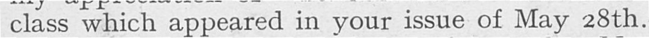class which appeared in your issue of May 28th.
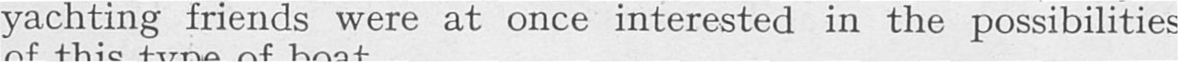yachting friends were at once interested in the possibilities
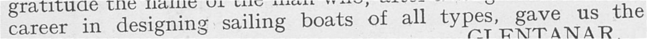career in designing sailing boats of all types, gave us the
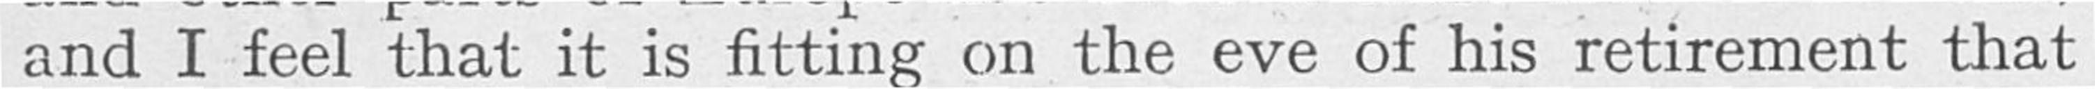and I feel that it is fitting on the eve of his retirement that
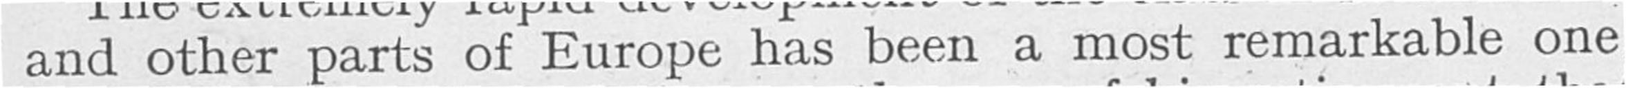and other parts of Europe has been a most remarkable one
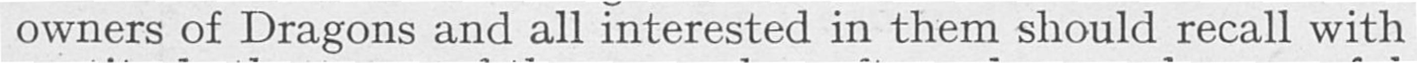owners of Dragons and all interested in them should recall with
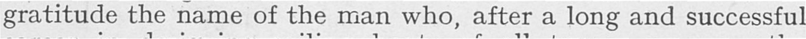gratitude the name of the man who, after a long and successful
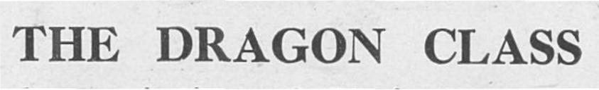THE DRAGON CLASS
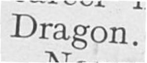Dragon.
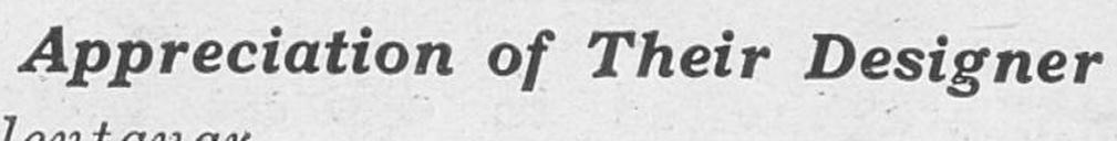Appreciation of Their Designer
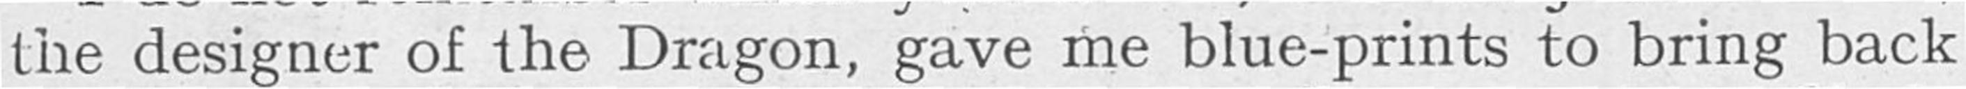the designer of the Dragon, gave me blue-prints to bring back
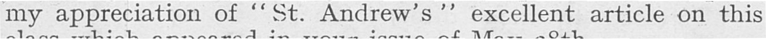my appreciation of "St. Andrew's" excellent article on this
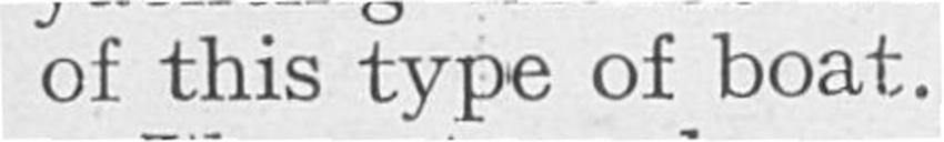of this type of boat.
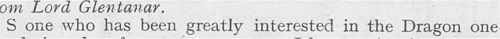As one who has been greatly interested in the Dragon one-
